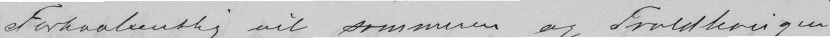Forhaabentlig vil sommeren og Troldhaugen
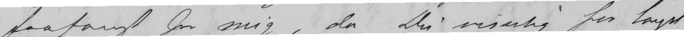faafængt for mig, da Du visselig for længst
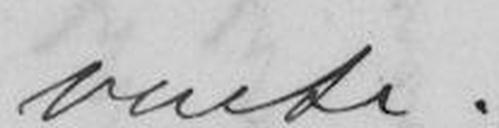vente.
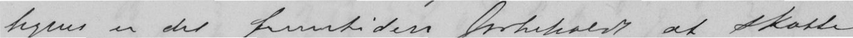ligens er det fremtiden forbiholdt at skatte
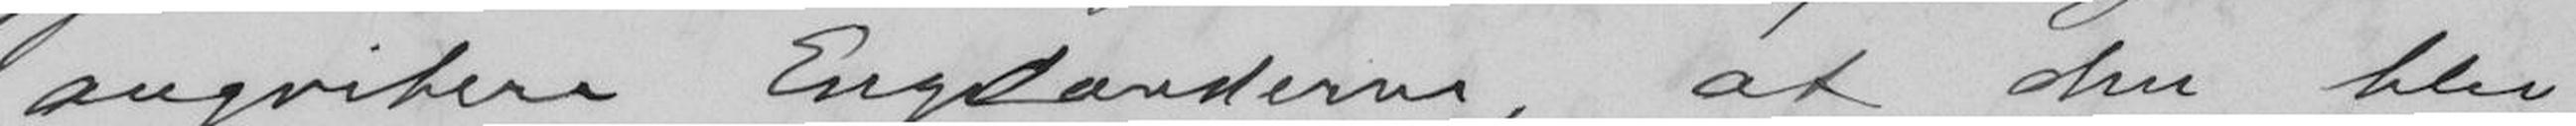angribere Englænderne, at den blev
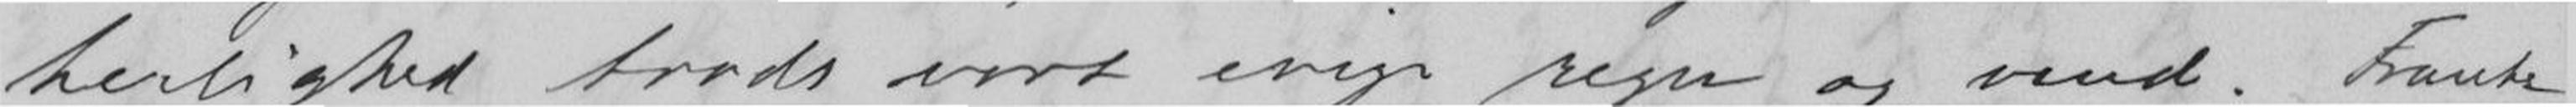herlighed trods vort evige regn og vind. Frantz
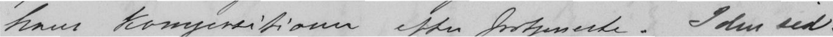hans kompositioner efter fortjeneste. I den sid-
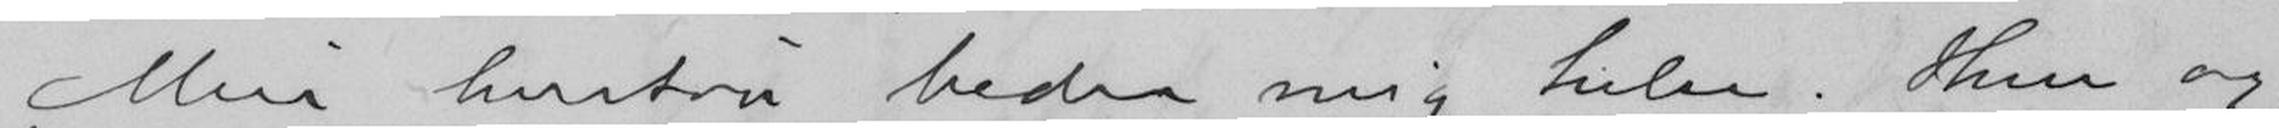Min hustru beder mig hilse. Hun og
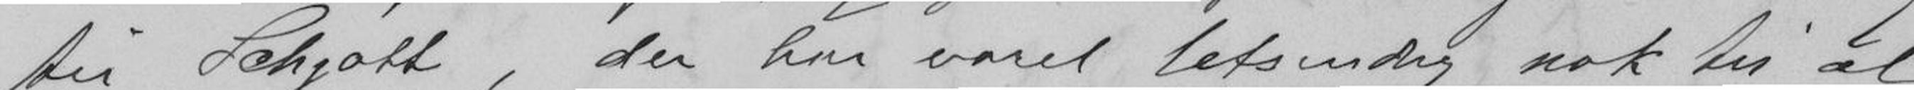til Schjøtt, der har været letsindig nok til at
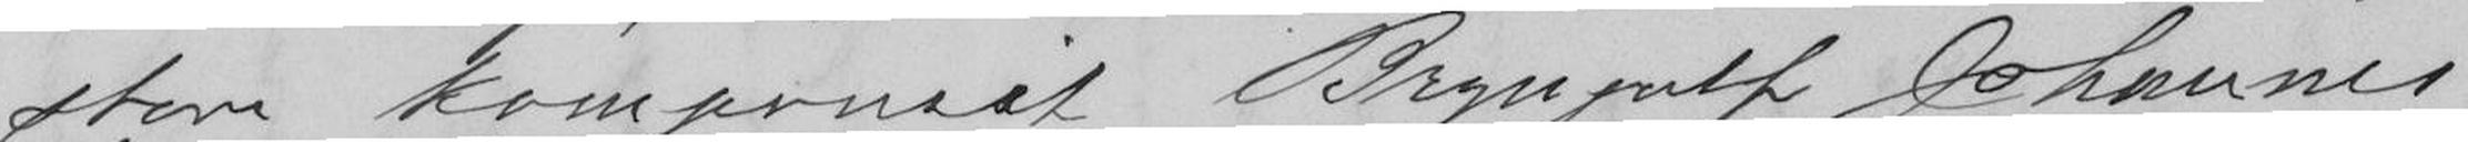store komponist Brynjulf Johannes-
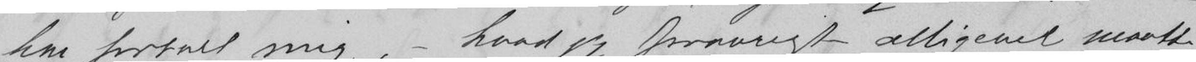har fortalt mig, - hvad jeg forøvrigt alligevel maatte
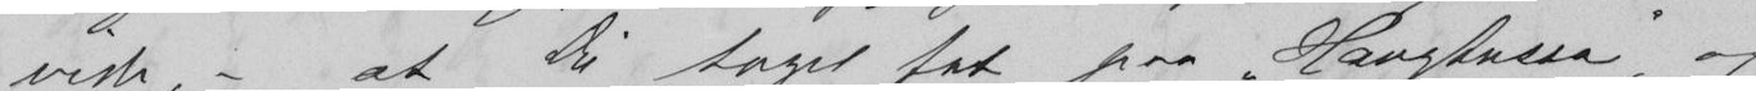vist, - at Du taget fat paa Haugtussa, og
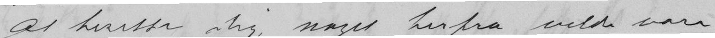At besette slig noget herfra vilde være
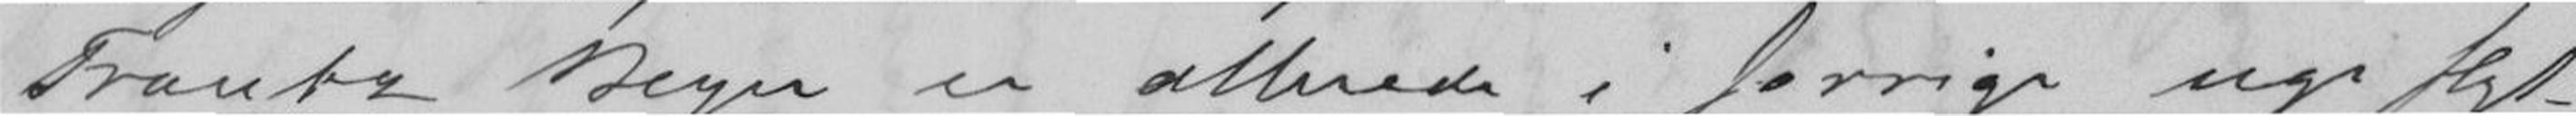Frantz Beyer er allerede i forrige uge flyt-
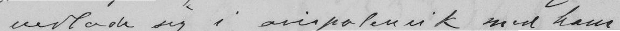indlade sig i avispolemik med ham.
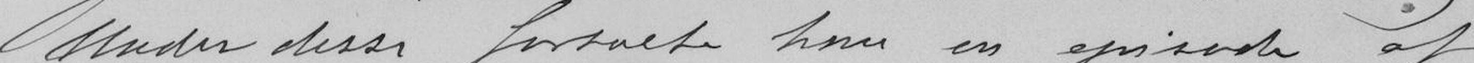Under disse fortalte han en episode af
